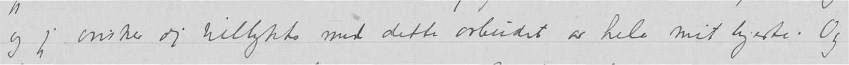og jeg ønsker deg tillykke med dette arbeidet av hele mit hjerte. Og
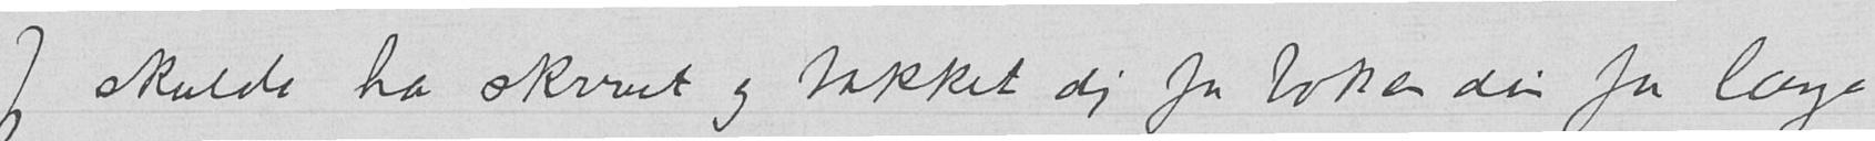Jeg skulde ha skrevet og takket dig for boken din for længe
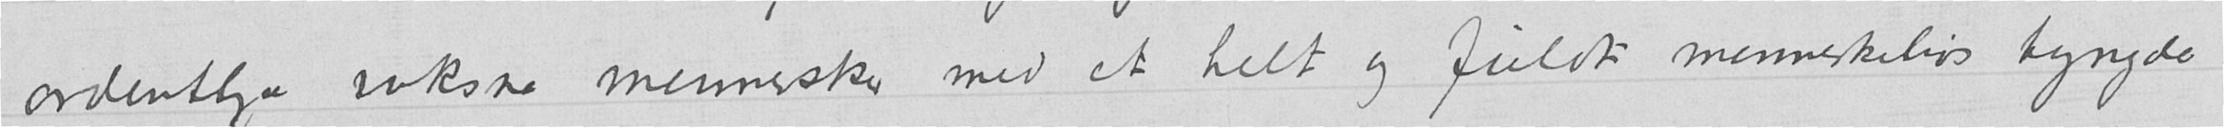ordentlige voksne mennesker med et helt og fuldt menneskelivs tyngde
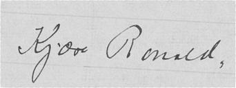Kjære Ronald,
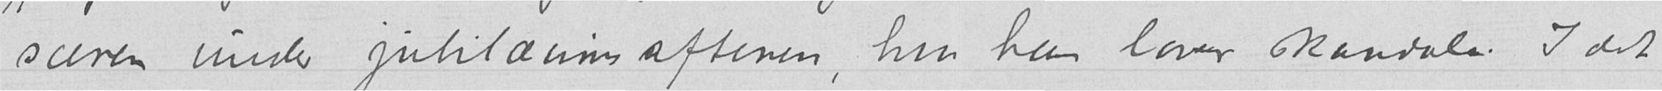scenen under jubilæumsaftenen, hvor han laver skandale. I det
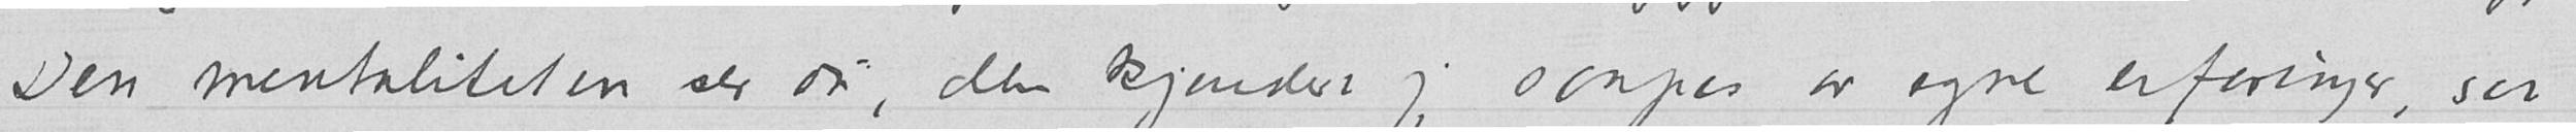Den mentaliteten ser du, den kjender jeg saapas av egne erfaringer, saa
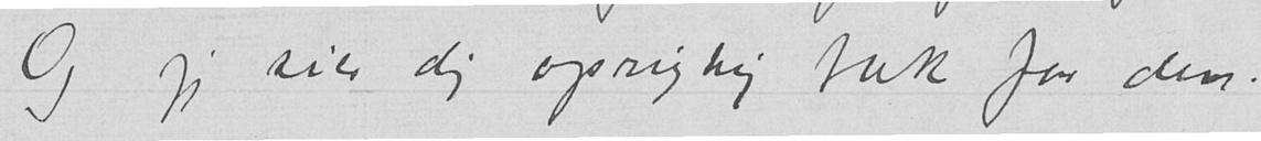Og jeg sier dig oprigtig tak for den.
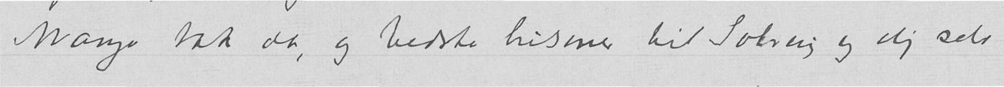Mange tak da, og bedste hilsener til Solveig og dig selv
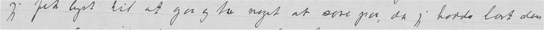jeg fik lyst til at gaa og ta noget at sove paa, da jeg hadde læst den
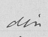din
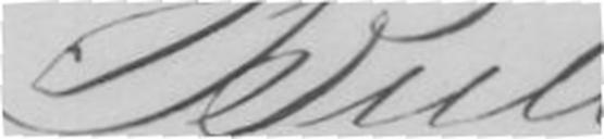Bull
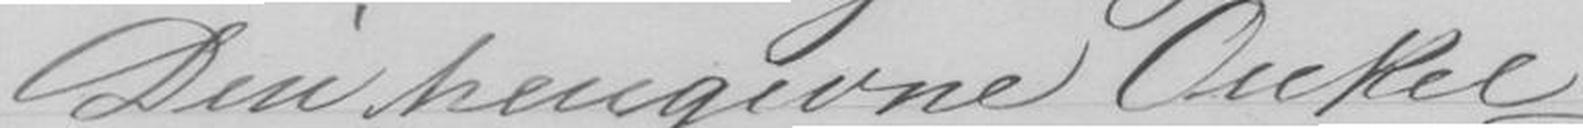Din hengivne Onkel
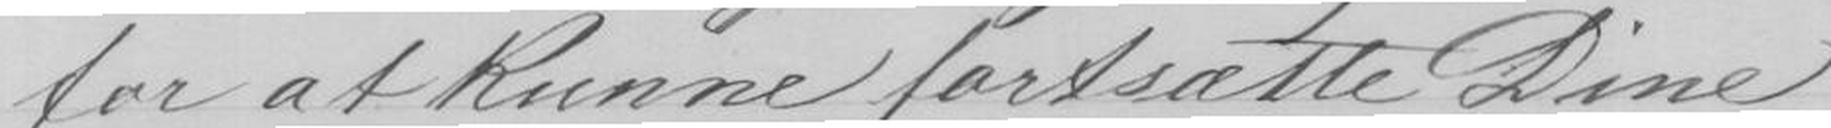for at kunne fortsætte Dine
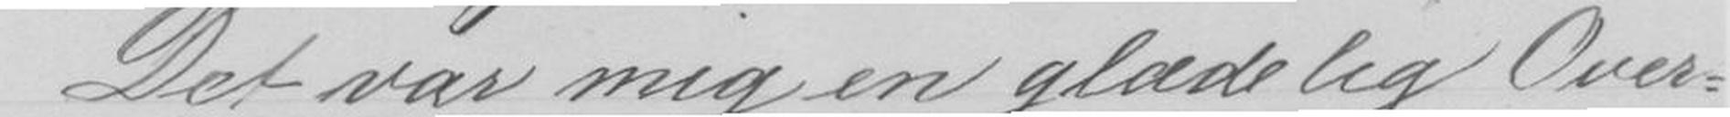Det var mig en glædelig Over-
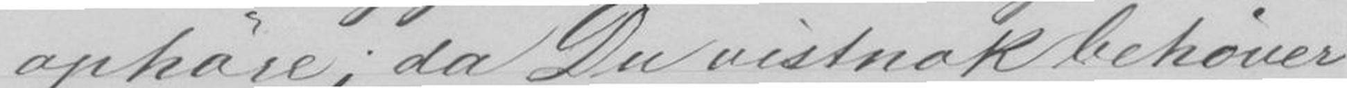ophøre; da Du vistnok behøver
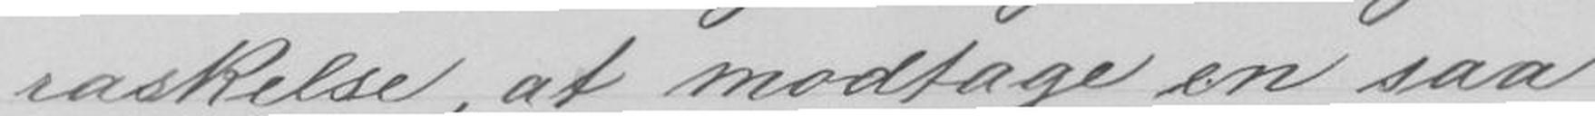raskelse, at modtage en saa
